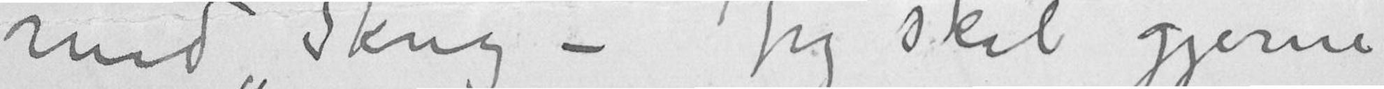med «Skrig» – Jeg skal gjerne
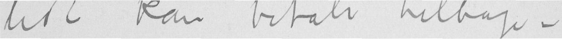lidt kan betale tilbage –
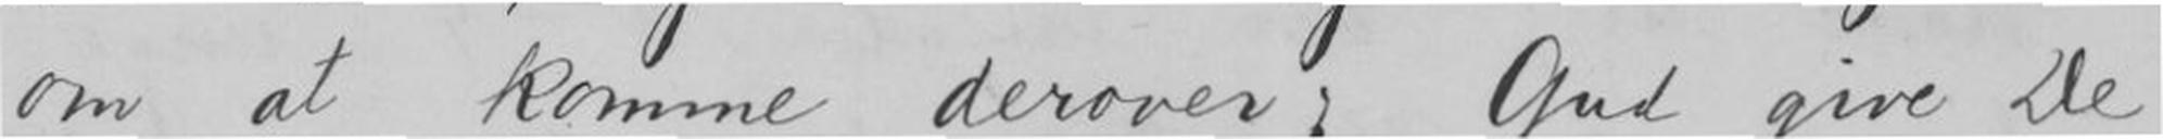om at komme derover, Gud give De
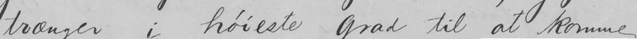trænger i høieste grad til at komme
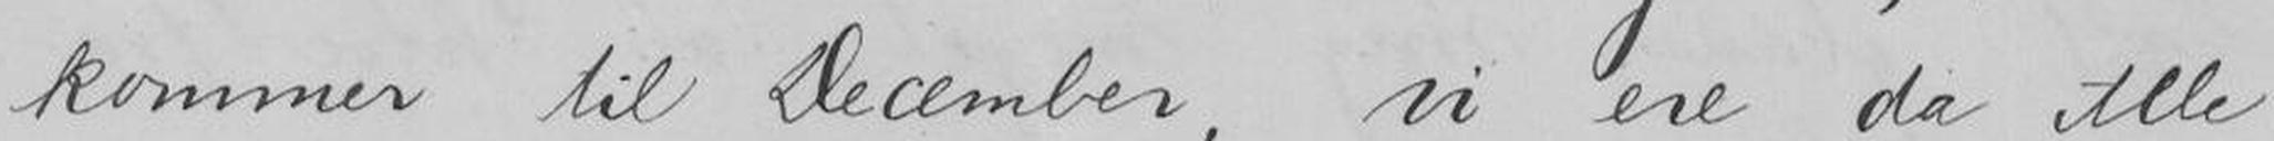kommer til December, vi ere da Alle
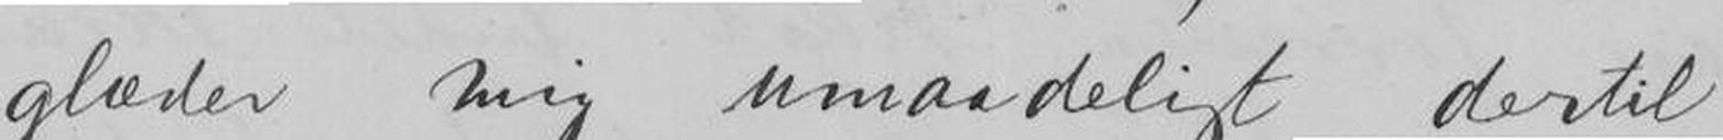glæder mig umaadeligt dertil, jeg
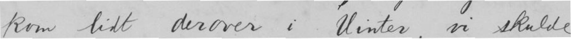kom lidt derover i Vinter, vi skulde
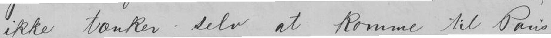ikke tænker selv at komme til Paris,
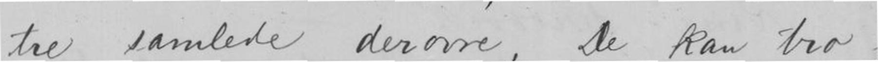tre samlede derovre, De kan tro jeg
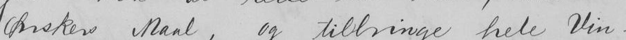Ønskers Maal, og tilbringe hele Vin-
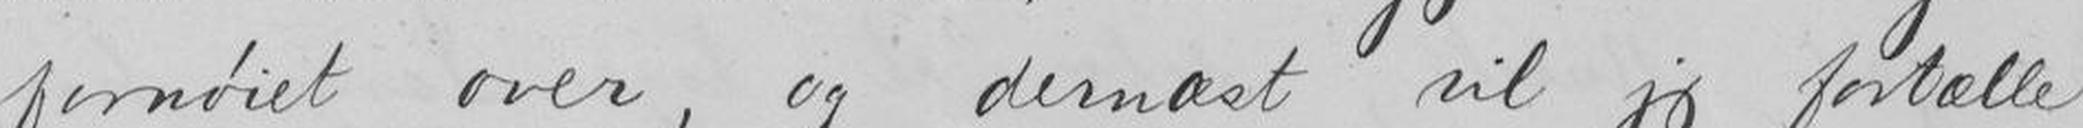fornøiet over, og dernæst vil jeg fortælle
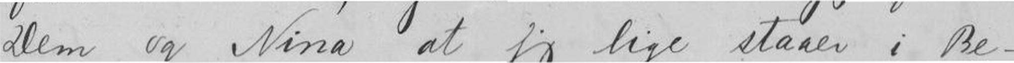Dem og Nina at jeg lige staaer i Be-
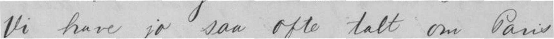Vi have jo saa ofte talt om Paris
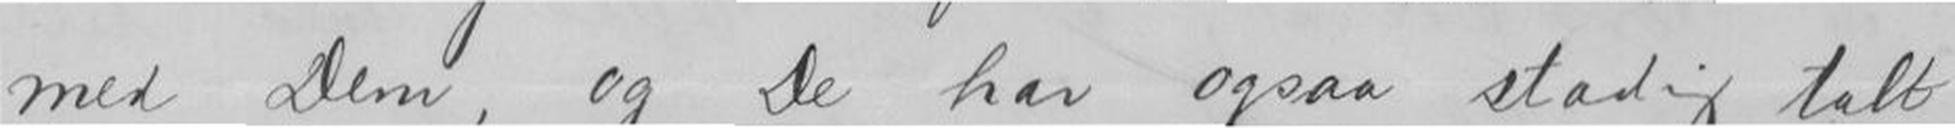med Dem, og De har ogsaa stadig talt
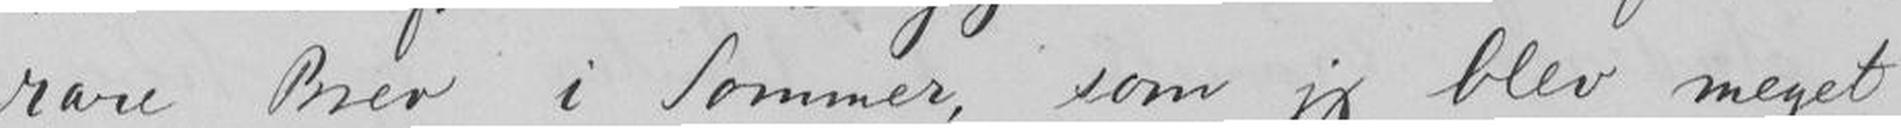rare Brev i Sommer, som jeg blev meget
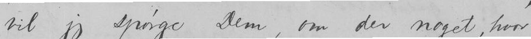vil jeg spørge Dem, om der noget, hvor
